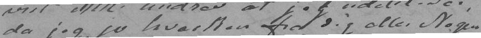da jeg jo hverken fra Dig eller Nogen
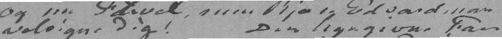velsigne Dig!Din hengivne Faer
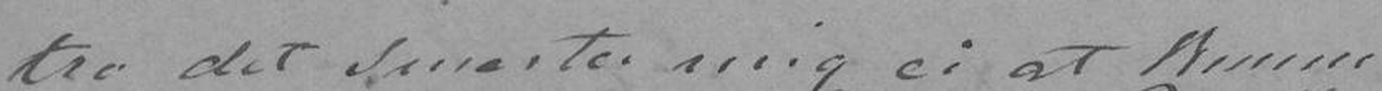tro det smerte mig ei at kunne
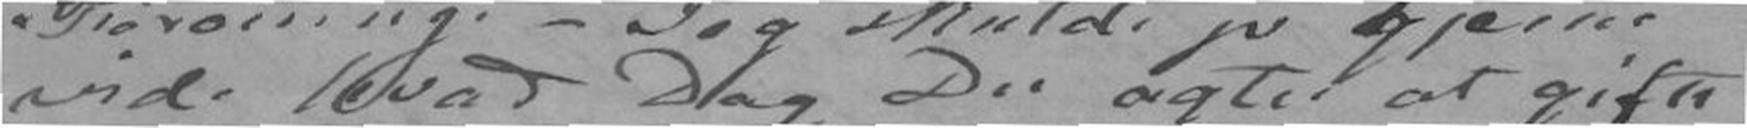vide hvad Dag Du agter at gifte
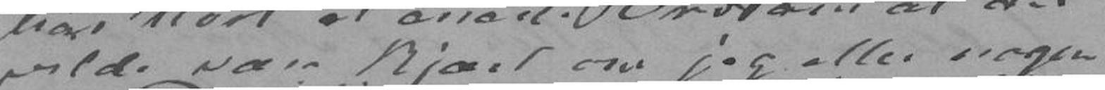vilde være kjært om jeg eller nogen
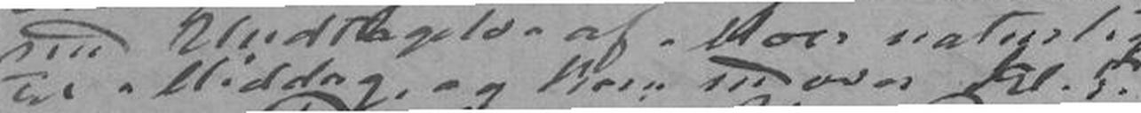til Middag, og kom indover Kl.5.
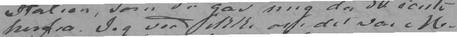herfra. Jeg veed ikke om det var Me-
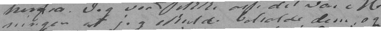ningen at jeg skulde beholde dem, og
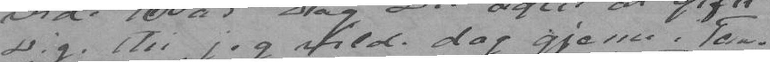Dig, thi jeg vilde dog gjerne i Tan-
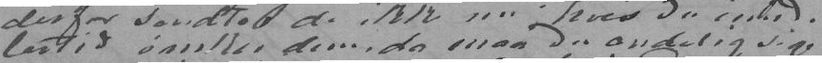lertid ønsker dem, da maa Du endelig sige
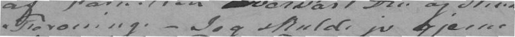Forening. - Jeg skulde jo gjerne
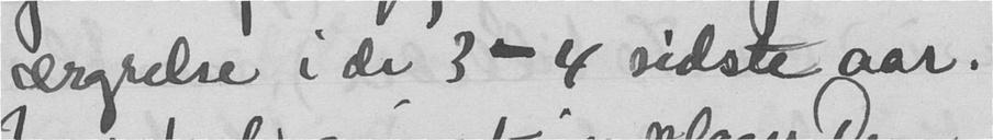ærgrelse i de 3-4 sidste aar.
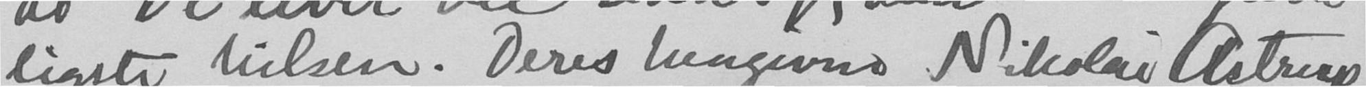ligste hilsen. Deres hengivne Nikolai Astrup
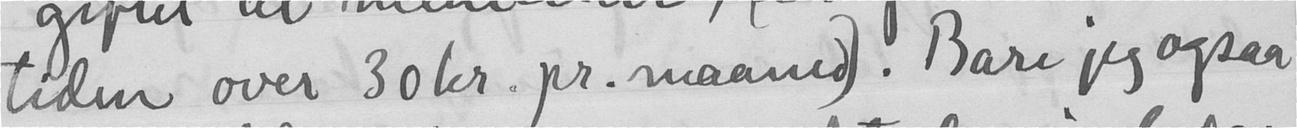tiden over 30kr. pr. maaned). Bare jeg ogsaa
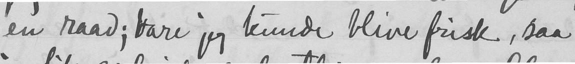en raad; bare jeg kunde blive frisk, saa
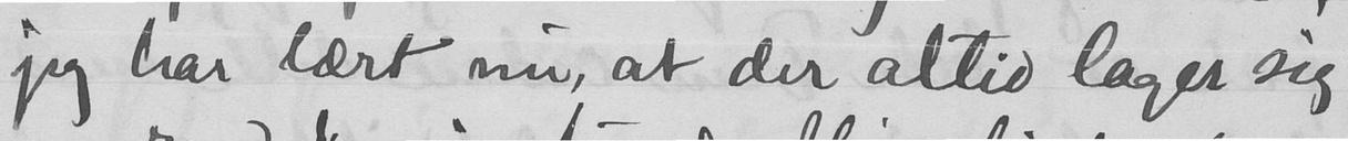jeg har dært nu, at der altid lager sig
